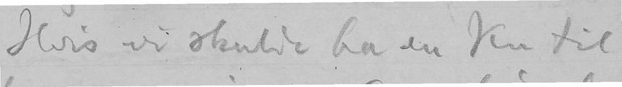Hvis vi skulde ha en Ku til
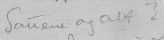Sauene og alt?
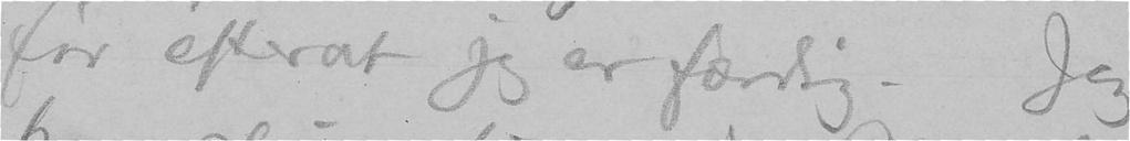før efterat jeg er færdig. Jeg
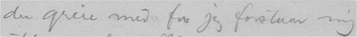du greie med for jeg forstaar mig
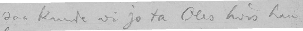saa kunde vi jo ta Oles hvis han
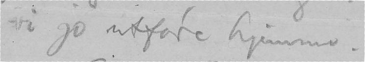vi jo utføre hjemme.
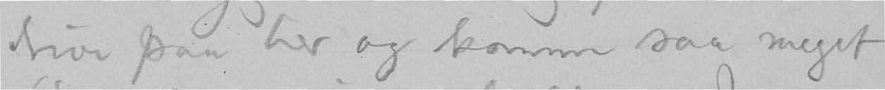drive paa her og komme saa meget
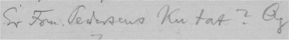Er Fru Pedersens Ku tat? Og
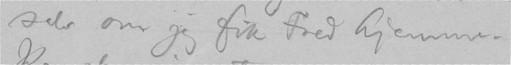selv om jeg fik Fred hjemme.
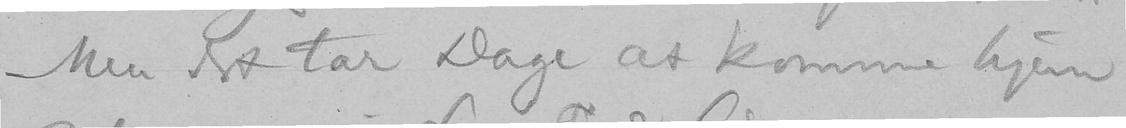Men det tar Dage at komme hjem
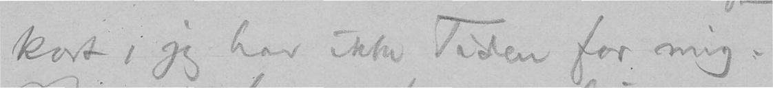kort, jeg har ikke Tiden for mig.
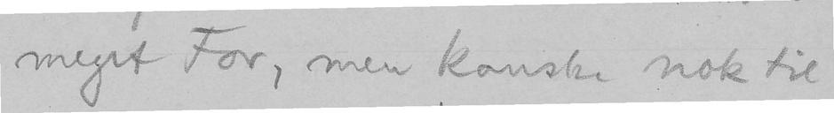meget For, men kanske nok til
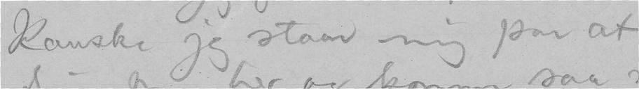Kanske jeg staar mig paa at
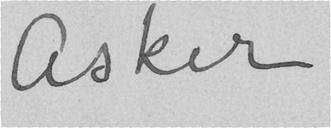Asker
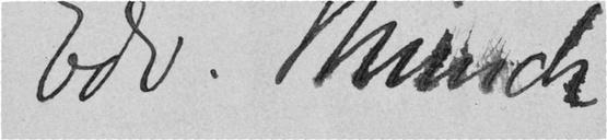Edv. Munch
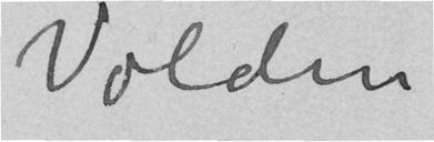Volden
